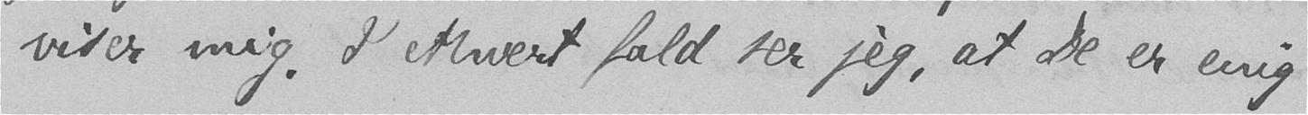viser mig. I ethvert fald ser jeg, at De er enig
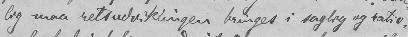lig maa retsudviklingen bringes i saglig og ratio-
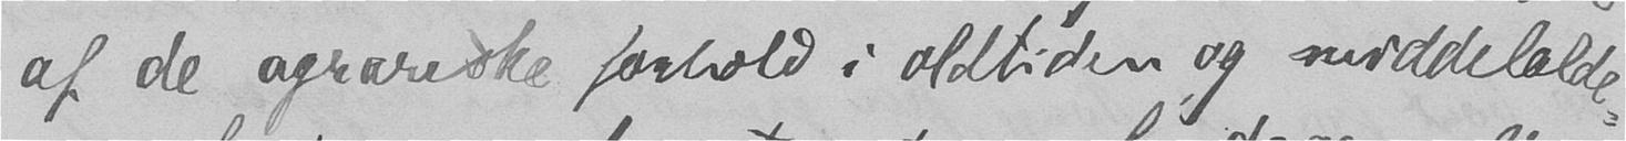af de agrariske forhold i oldtiden og middelalde-
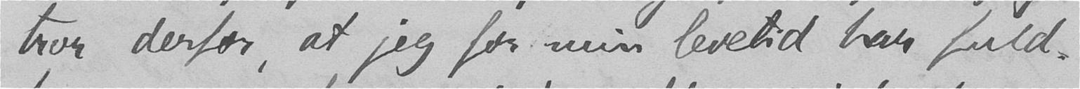tror derfor, at jeg for min levetid har fuld-
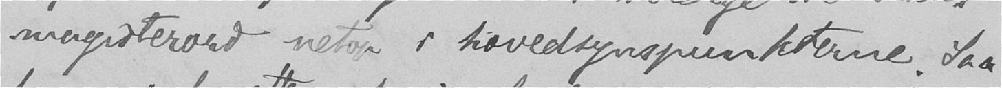magisterord netop i hovedsynspunkterne. Saa
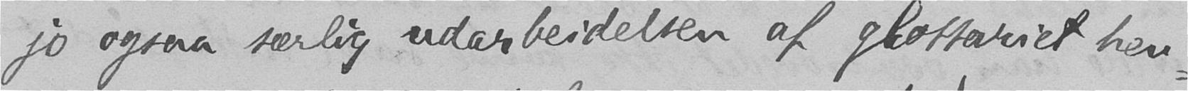jo ogsaa særlig udarbeidelsen af glossariet hen-
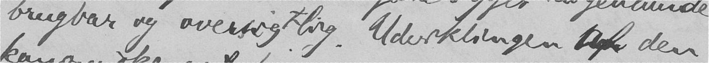brugbar og oversigtlig. Udviklingen af den
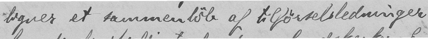ligner et sammenløb af tilførselsledninger
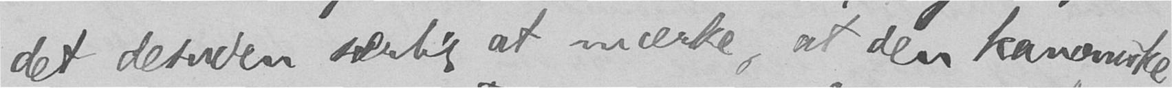det desuden særlig at mærke, at den kanoniske
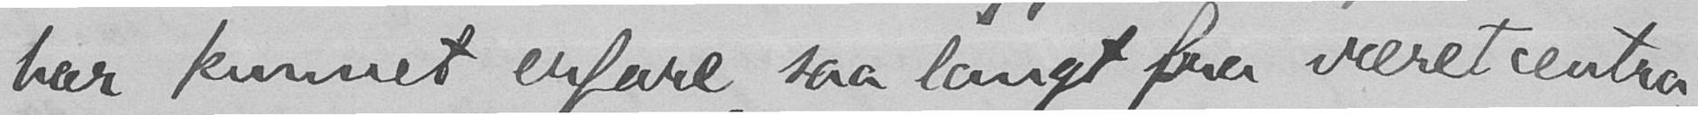har kunnet erfare, saa langt fra været centra-
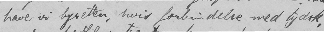have vi byretten, hvis forbindelse med tydsk,
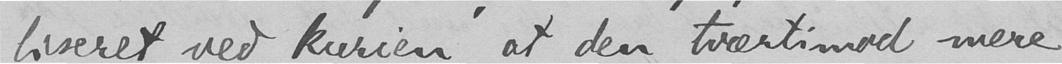liseret ved kurien, at den tværtimod mere
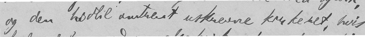og den hidtil omtrent uskrevne kirkeret, hvis
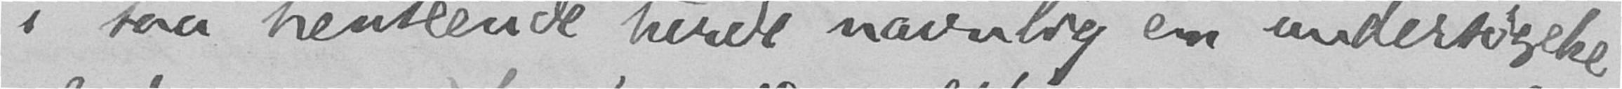i saa henseende turde navnlig en undersøgelse
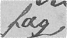fag
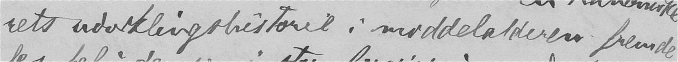rets udviklingshistore i middelalderen fremde-
